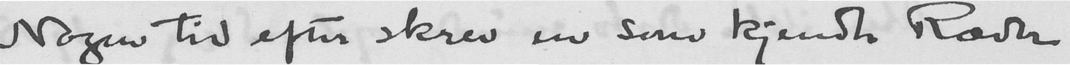Nogen tid efter skrev en som kjende Ræder
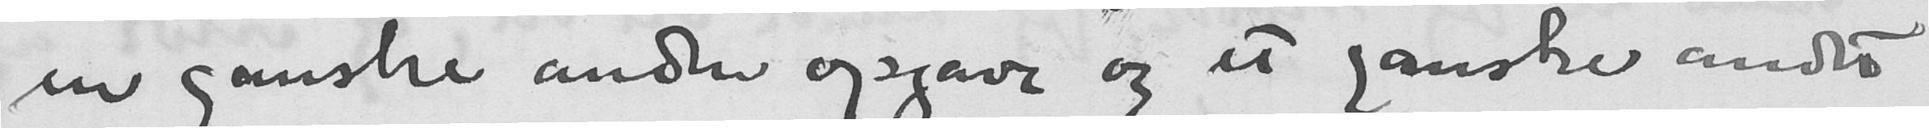en ganske anden opgave og et ganske andet
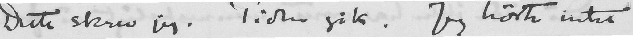Dette skrev jeg. Tiden gik. Jeg hørte intet
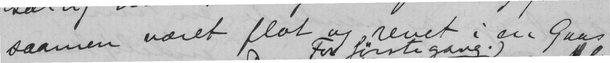saamen været flot og revet i en Gaas
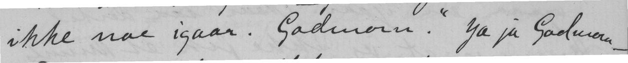ikke noe igaar. Godmorn." Ja ja Godmorn-
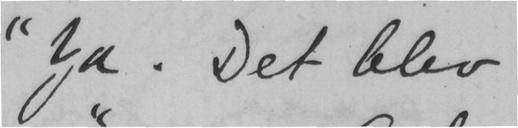"Ja. Det blev
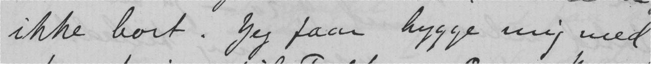ikke bort. Jeg faar hygge mig med
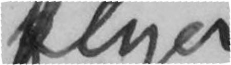flyer
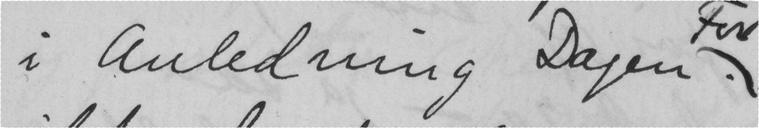i Anledning Dagen.
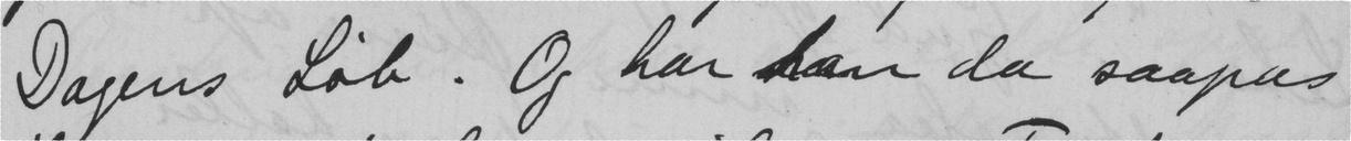Dagens Løb. Og har han da saapas
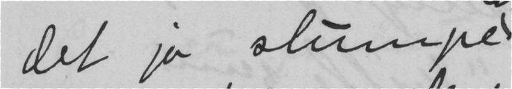det jo slumpe
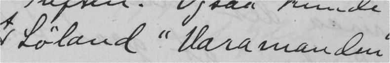Løland "Varamanden"
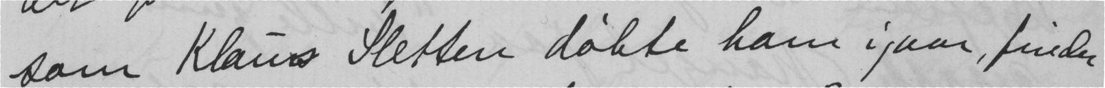som Klaus Sletten døbte ham igaar, finder
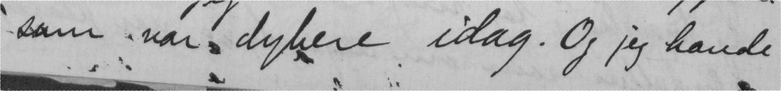som var dybere idag. Og jeg havde
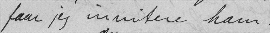faar jeg invitere ham.
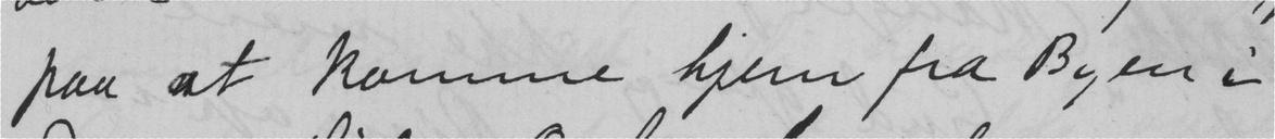paa at komme hjem fra Byen i
